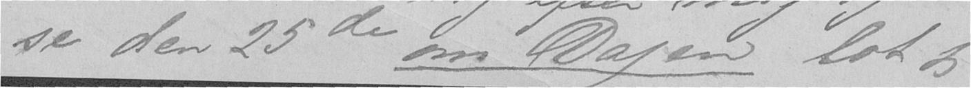se den 25de om Dagen lot jeg
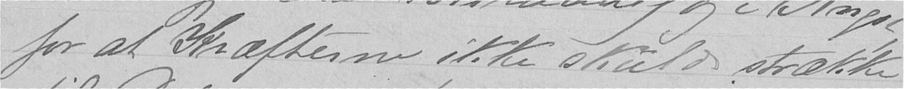for at Kræfterne ikke skulde strække
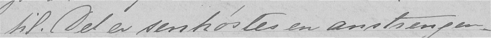til. Det er senhøstes en anstrengen-
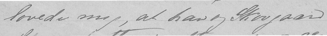lovede mig, at han og Skovgaard
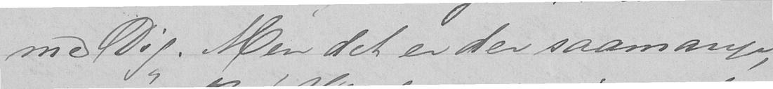med Dig. Men det er der saamange
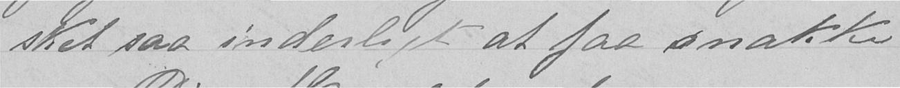sket saa inderligt at faa snakke
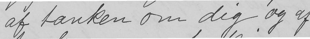af tanken om dig og af
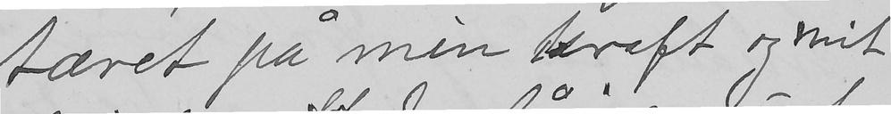tæret på min kraft og mit
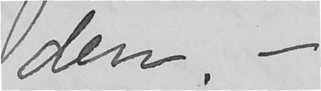den. -
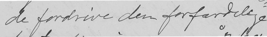de fordrive den forfærdelige
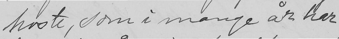hoste, som i mange år har
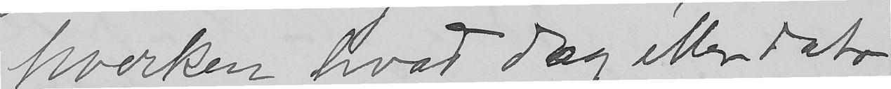hverken hvad dag eller dato
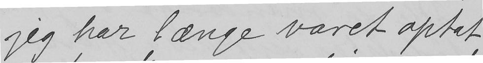jeg har længe været optat
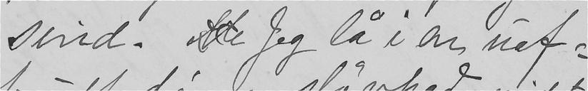sind. Me Jeg lå i en uaf-
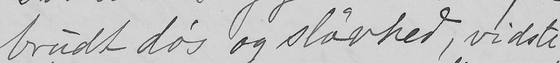brudt døs og sløvhed, vidste
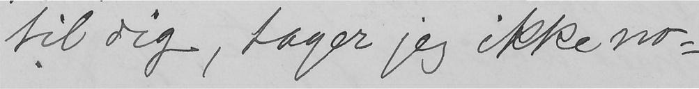til dig, tager jeg ikke no-
